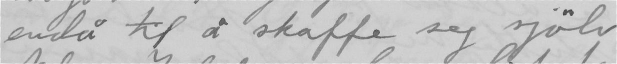endå til å skaffe seg sjölv
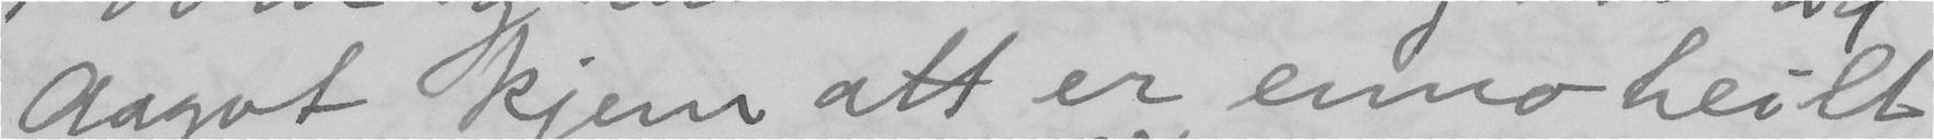Aagot kjem att er enno heilt
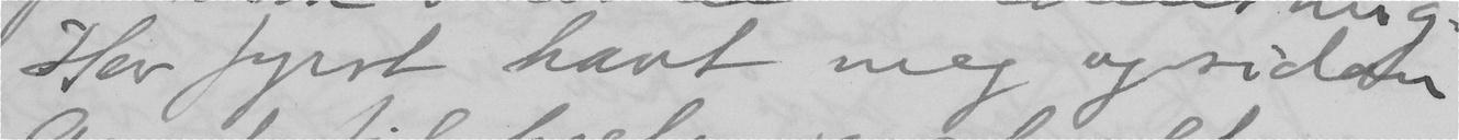Hev fyrst havt meg og sidan
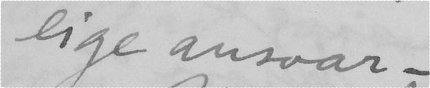eige ansvar –
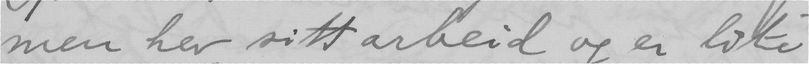men hev sitt arbeid og er lite
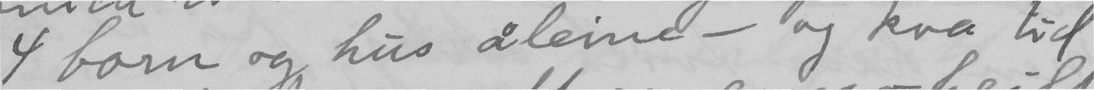4 born og hus åleine – og kva tid
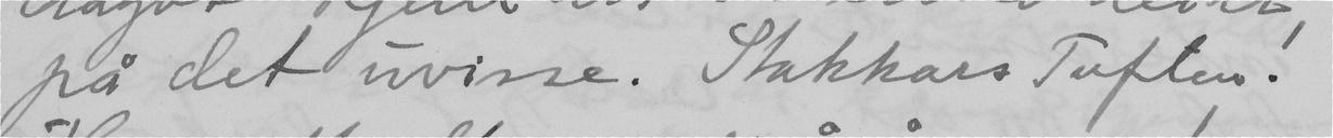på det uvisse. Stakkars Tuften!
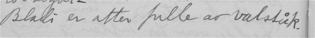Bladi er atter fulle av valståk.
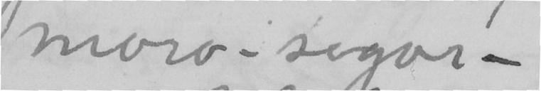moro-sogor –
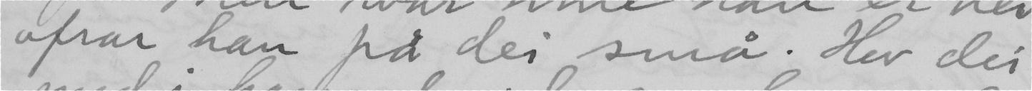ofrar han på dei små. Hev dei
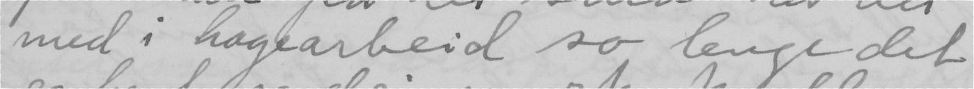med i hagearbeid so lenge det
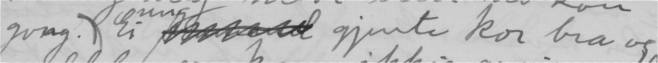gong. Ei ung  gjente kor bra og
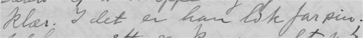klær. I det er han lik far sin.
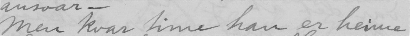Men kvar time han er heime
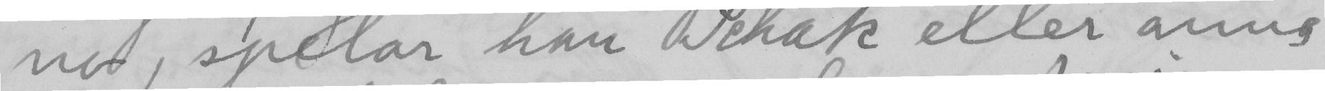no, spelar han schak eller anna
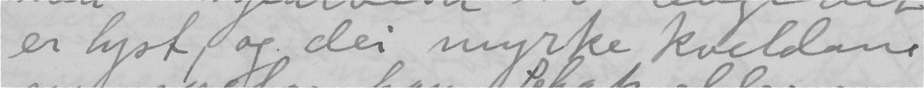er lyst, og dei myrke kveldane
